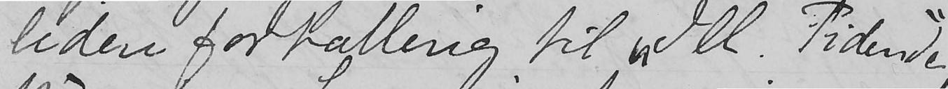liden fortælling til "Ill. Tidende",
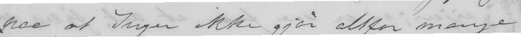paa at Ingen ikke gjør alfor mange
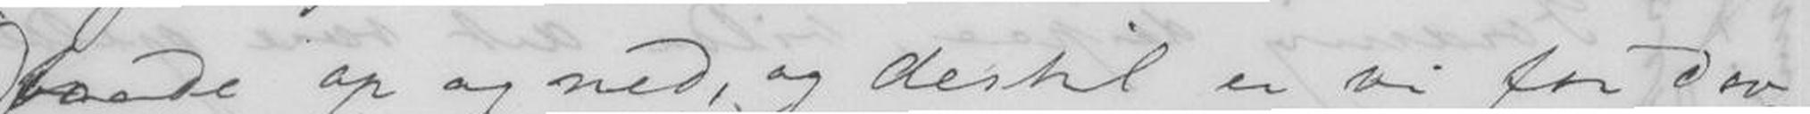baade op og ned, og dertil er vi for dov-
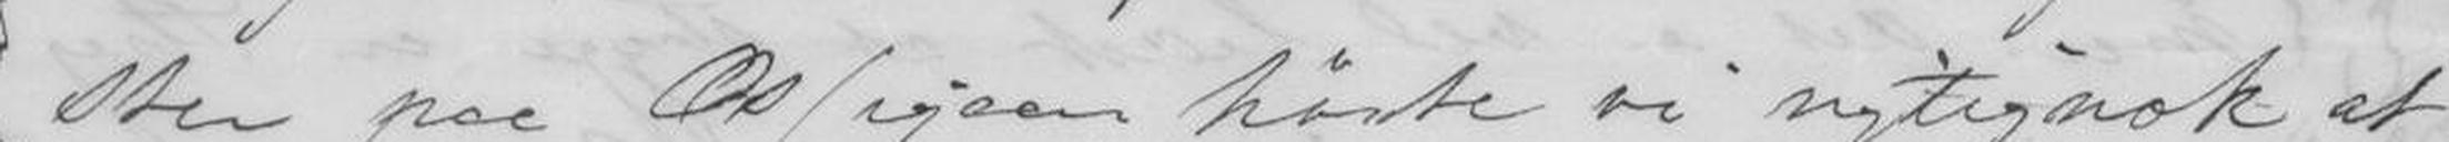ster paa Os (igaar hørte vi rigtignok at
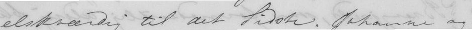elskværdig til det Sidste; Johanne og
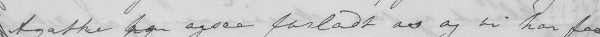Agathe har ogsaa forladt os og vi har faa-
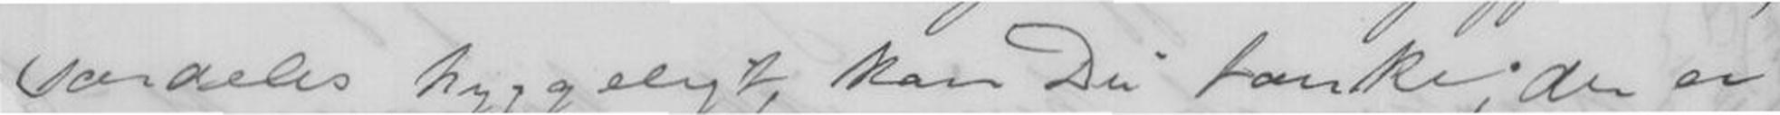særdeles hyggeligt, kan Du tænke; der er
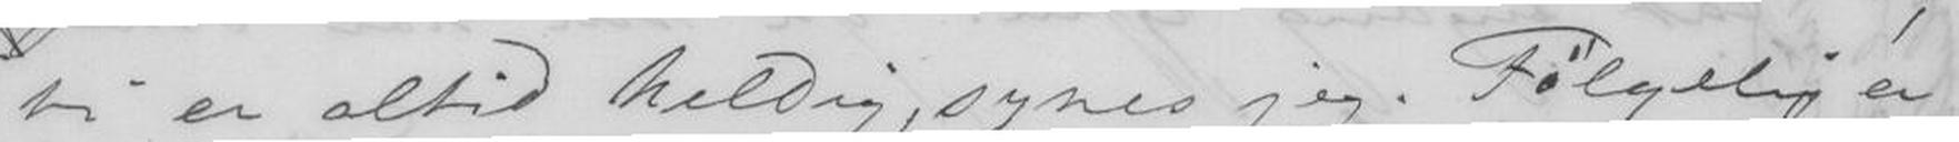vi er altid heldig, synes jeg. Følgelig er
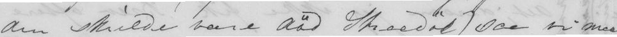den skulde være død Streedøl) saa vi maa
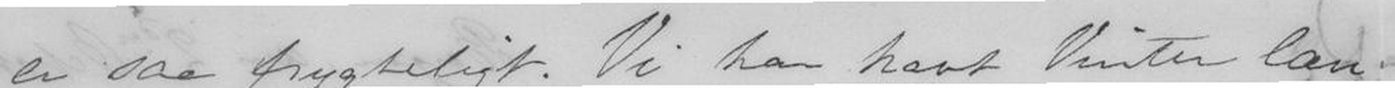er saa frygteligt. Vi har havt Vinter læn-
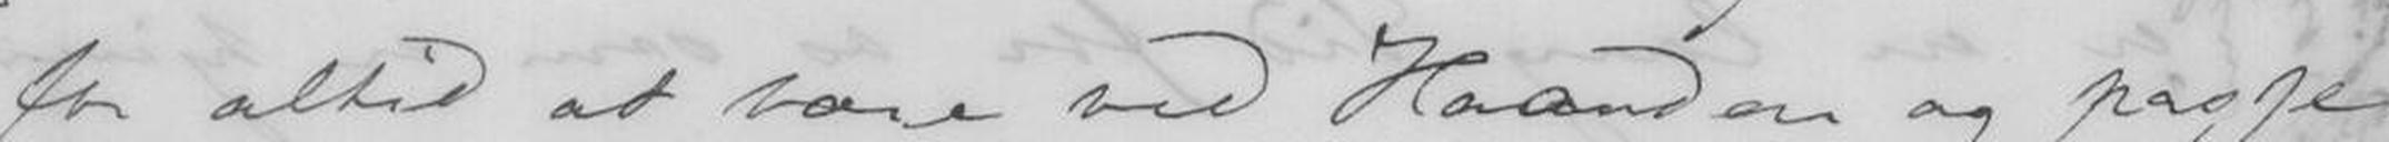for altid at være ved Haanden og passe
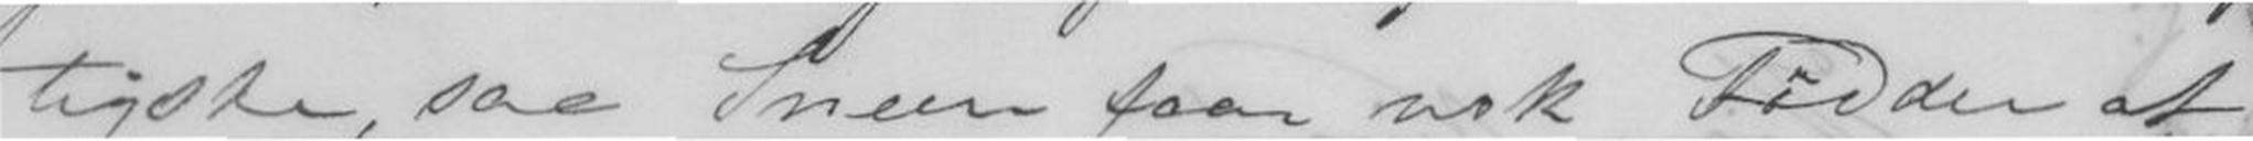tigste, saa Sneen faar nok Fødder at
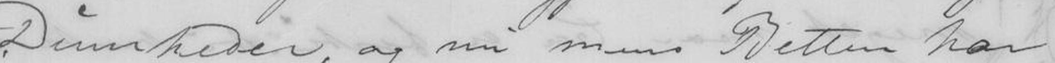Dumheder, og nu mens Betten har
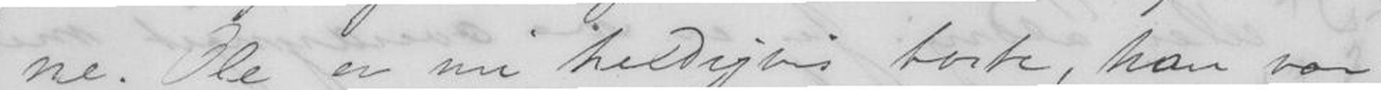ne. Ole er nu heldigvis borte, han var
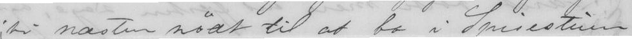vi næsten nødt til at bo i Spisestuen
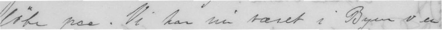løbe paa. Vi har nu været i Byen i en
